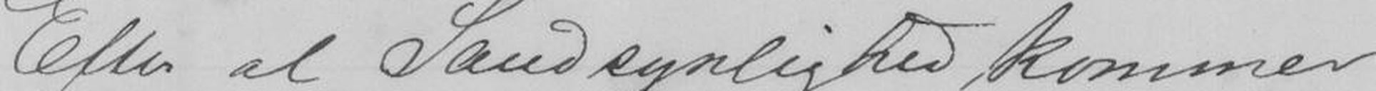Efter al Sandsynlighed kommer
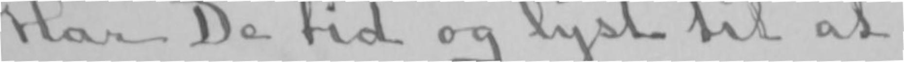Har De tid og lyst til at
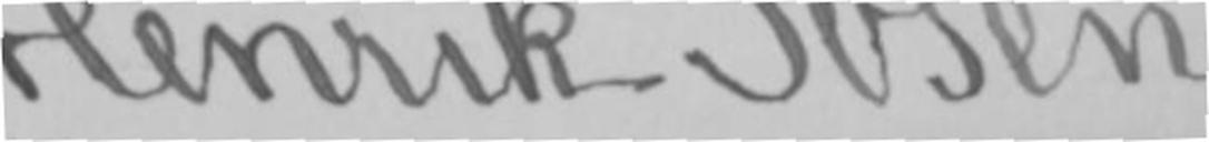Henrik Ibsen.
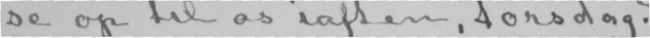se op til os iaften, torsdag?
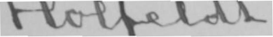Holfeldt.
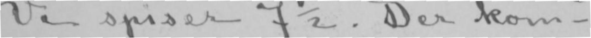Vi spiser 7½. Der kom-
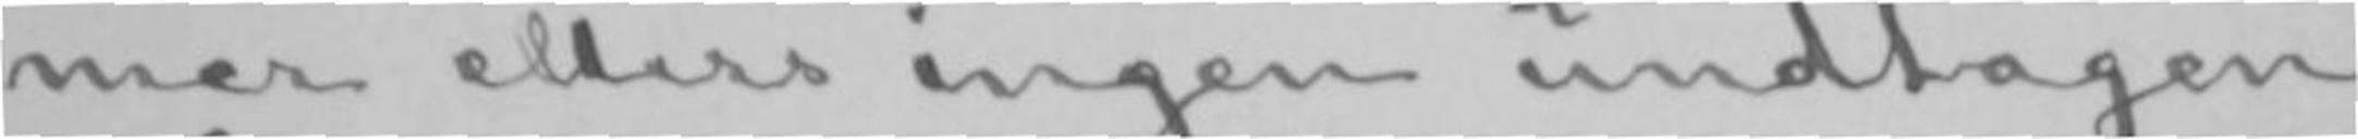mer ellers ingen undtagen
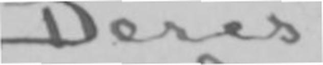Deres
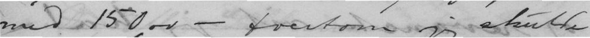med 150,00 - tvertom, jeg skulde
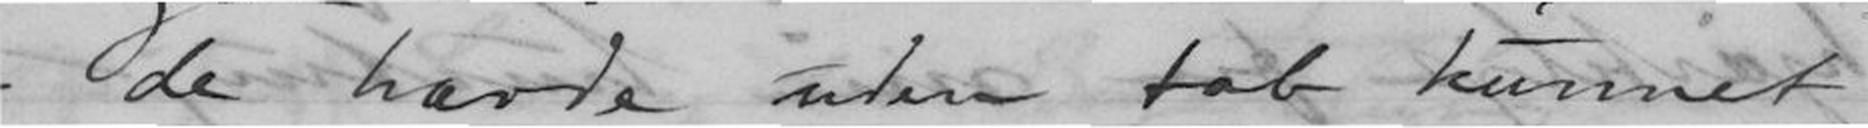- de havde uden tab kunnet
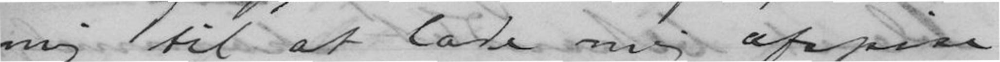mig til at lade mig afspise
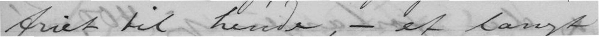friet til hende, - et langt
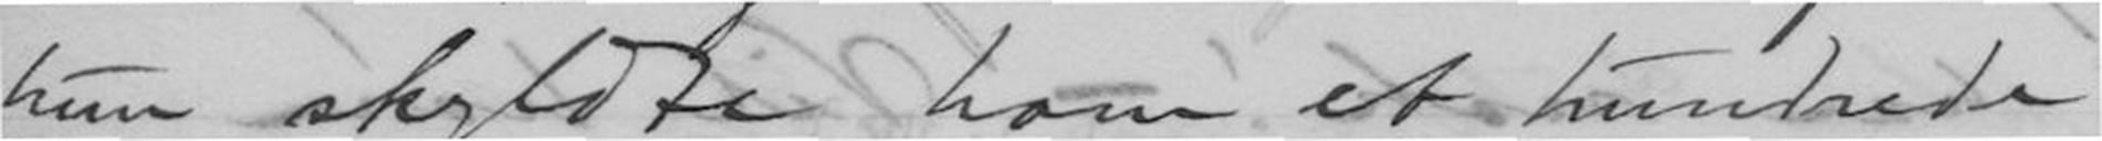hun skyldte ham et hundrede
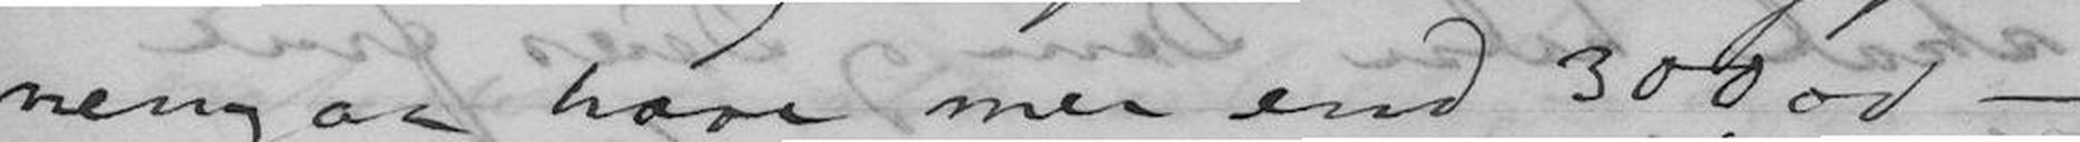nemgaa have mer end 300,00 -
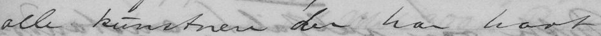alle kunstnere der har havt
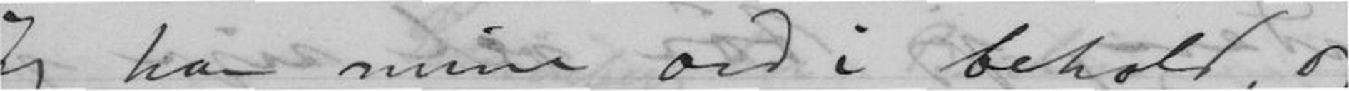Jeg har mine ord i behold, og
